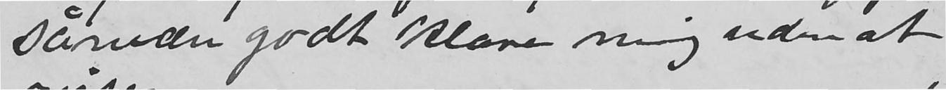såmæn godt klare mig uden at
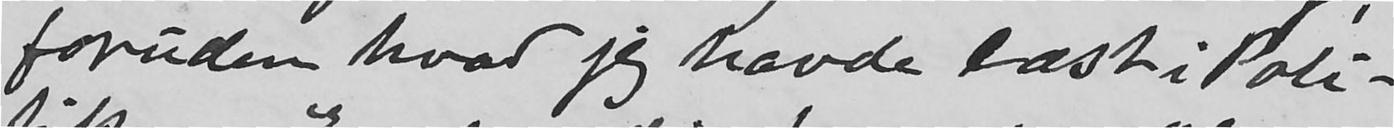foruden hvad jeg havde læst i Poli-
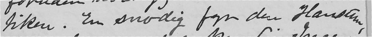tiken. En snodig fyr den Hamsun,
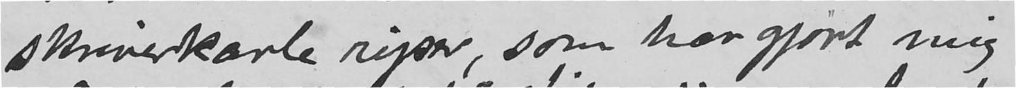skriverkarle rejser, som har gjort mig
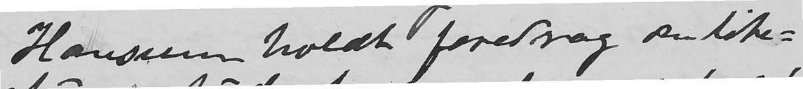Hamsun holdt foredrag om lite-
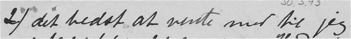2) det bedst at vente med til jeg
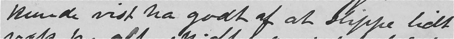kunde vist ha godt af at slippe lidt
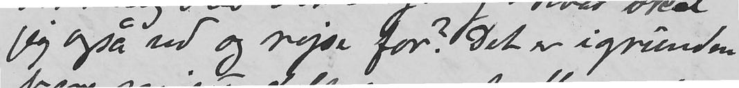jeg også ved og rejse for? Det er igrunden
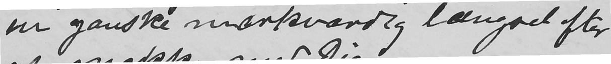en ganske mærkværdig længsel efler
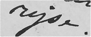rejse.
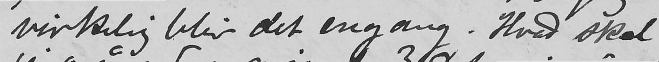virkelig blir det engang. Hvad skal
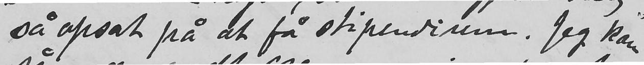så opsat på at få stipendium. Jeg kan
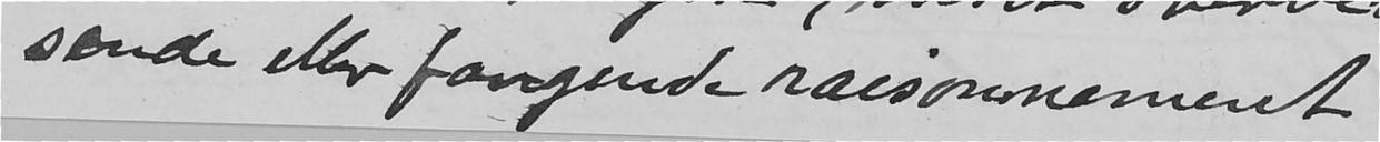sende eller fængende raisonnement
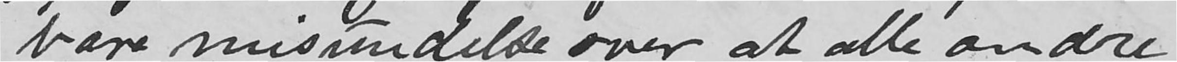bare misundelse over at alle andre
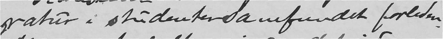ratur i studentersamfundet forleden.
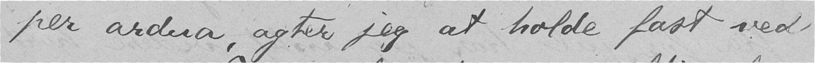per ardua, agter jeg at holde fast ved
